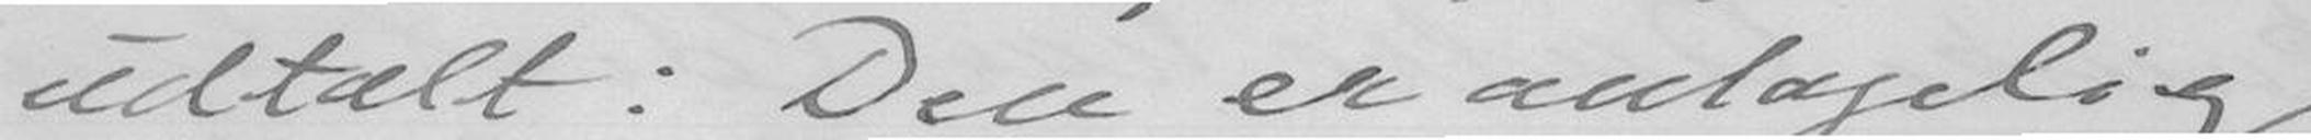udtalt: Den er antagelig
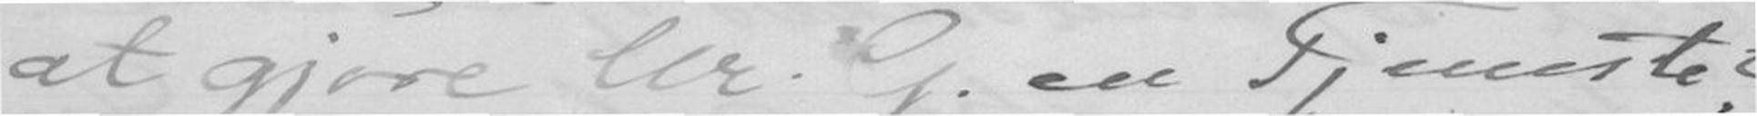at gjøre hr. G. en Tjeneste.
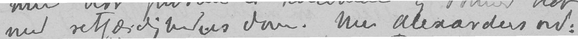med retfærdighedens dom. Nu Alexanders ord:
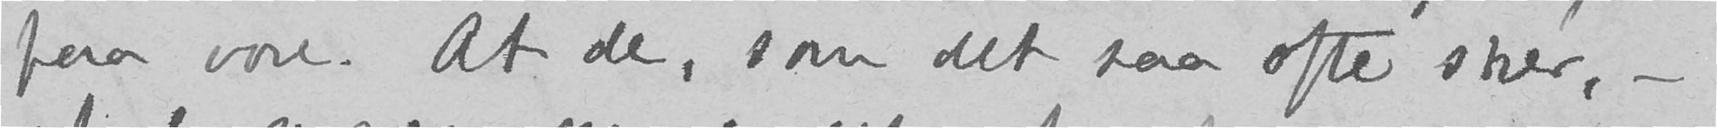paa vore. At de, som det saa ofte sker, -
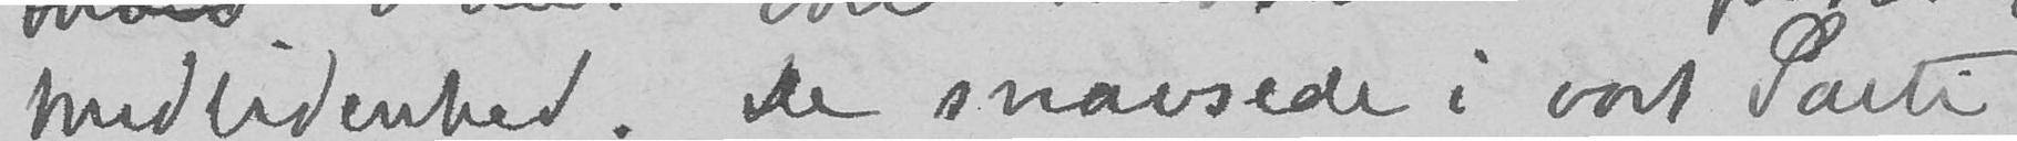medlidenhed. De snavsede i vort Parti
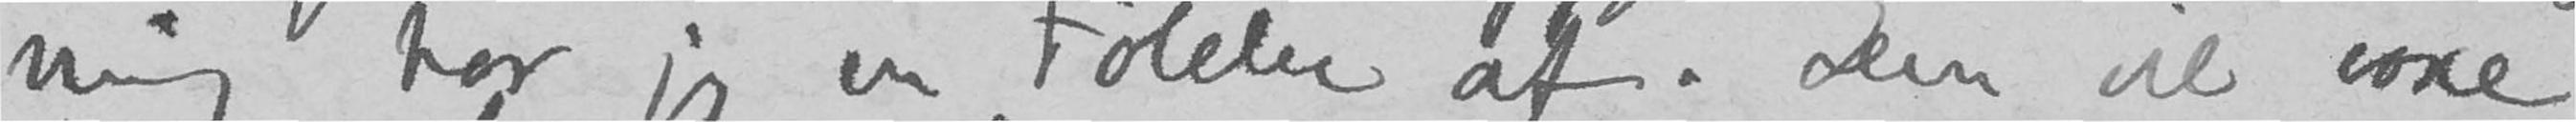ning har jeg en Følelse af. Den vil voxe
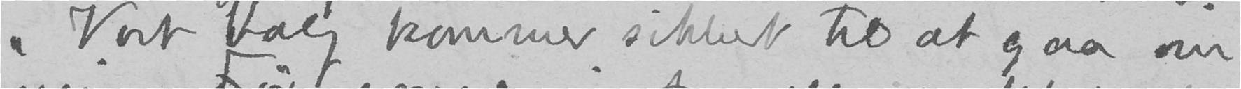Vort Valg kommer sikkert til at gaa om
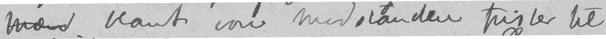mænd blant vore modstandere frister til
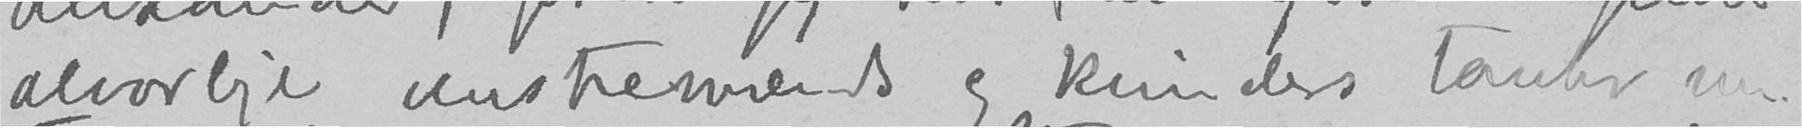alvorlige venstremænds og kvinders tanker nu.
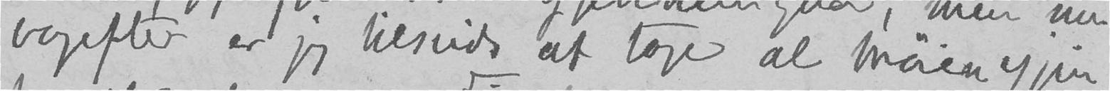bagefter, er jeg tilvist at tage al møie igjen
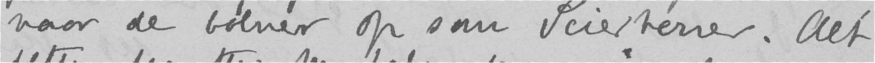naar de bolner op som Seierherrer. Alt
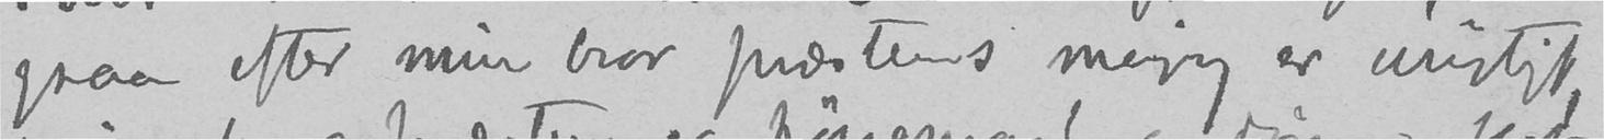ogsaa efter min bror præstens mening er urigtigt.
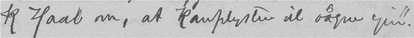Haab om, at Kamplysten vil vågne igjen".
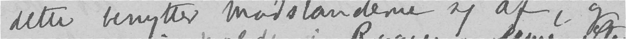dette benytter Modstanderne sig af, og
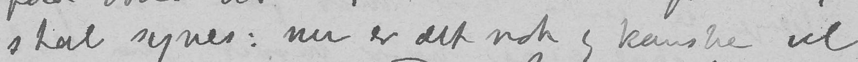skal synes: nu er det nok og kanske vel
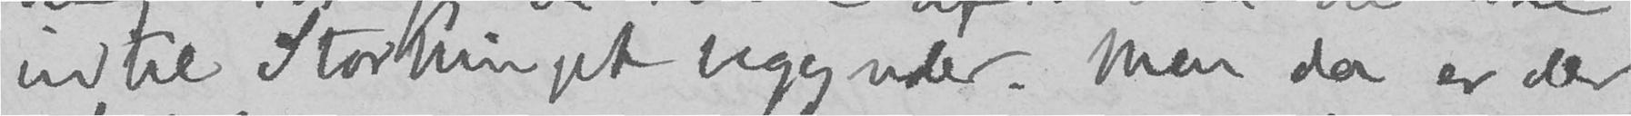indtil Stortinget begynder. Men da er der
